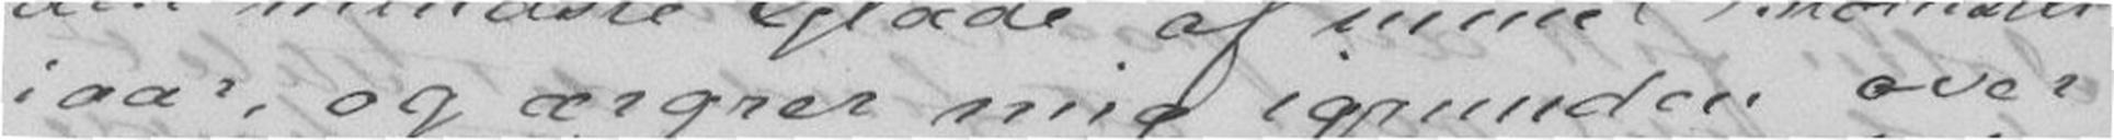iaar, og ærgrer mig igrunden over
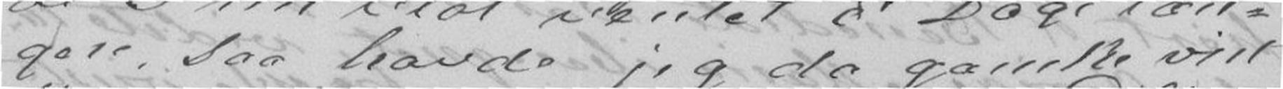gere, saa havde jeg da ganske vist
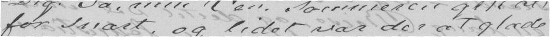for snart, og lidet var der at glæde
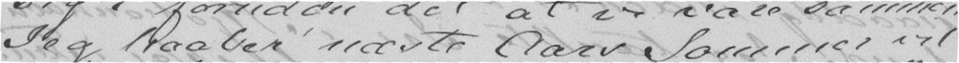Jeg haaber næste Aars Sommer vil
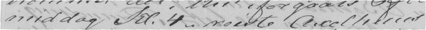middag Kl.4 reiste Axelhuus
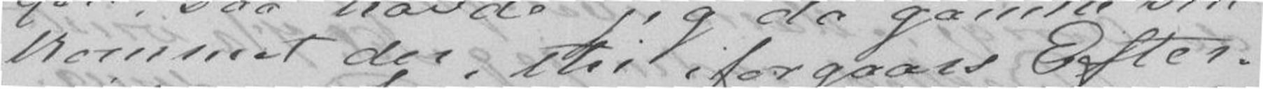kommet der, thi iforgaars Efter-
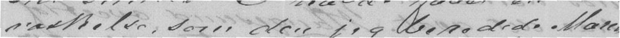raskelse, som den jeg beredede Maren,
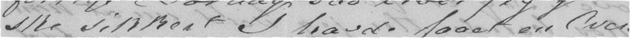ske sikkert I havde faaet en Over-
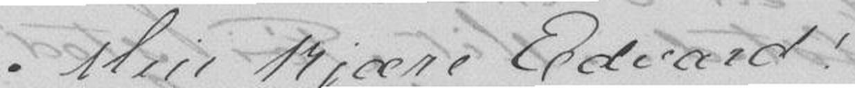Min kjære Edvard!
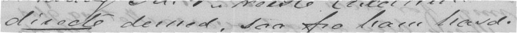directe derned, saa fra ham havde
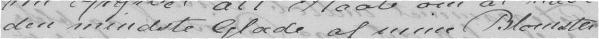den mindste Glæde af mine Blomster
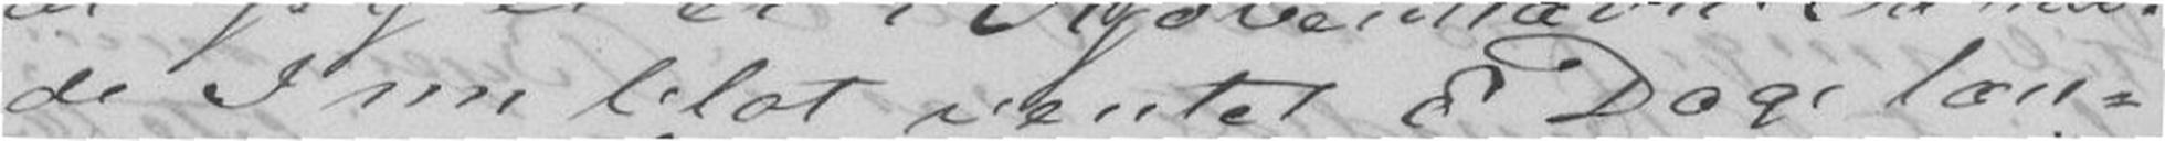de I nu blot ventet 8 Dage læn-
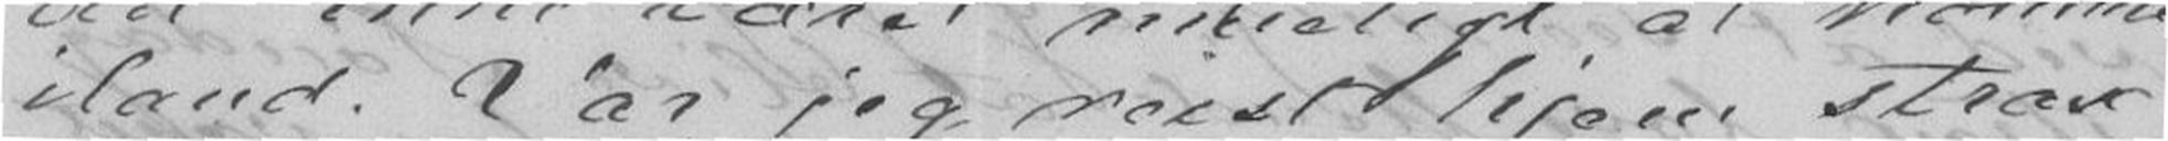iland, Var jeg reist hjem strax
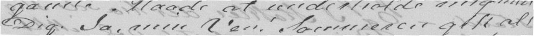Dig. Ja, min Ven! Sommeren gik alt
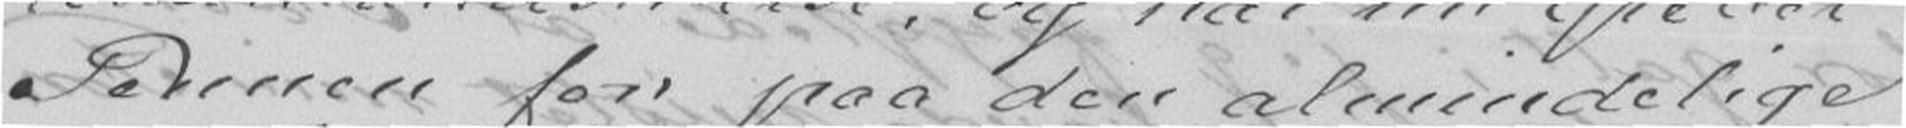Pennen for paa den almindelige
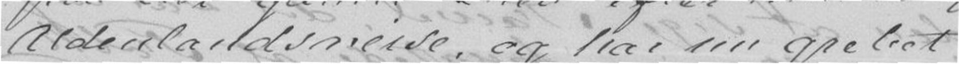Udenlandsreise, og har nu grebet
